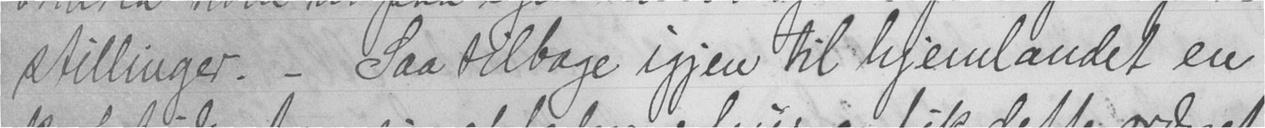stillinger. - Saa tilbage igjen til hjemlandet en
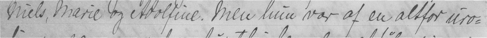Niels, Marie og Adolfine. Men hun var af en altfor uro-
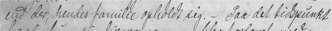end der, hendes familie opholdt sig. - Paa det tidspunkt
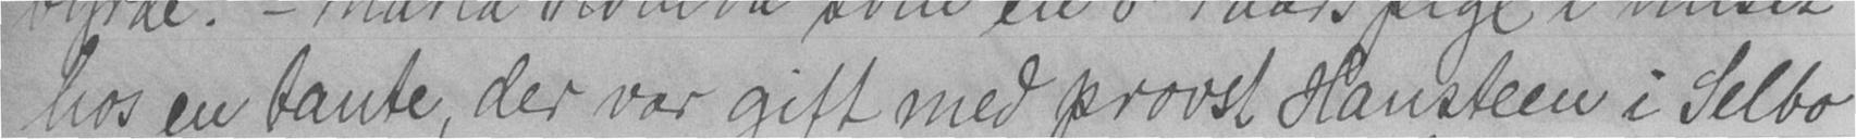hos en tante, der var gift med provst Hansteen i Selbo
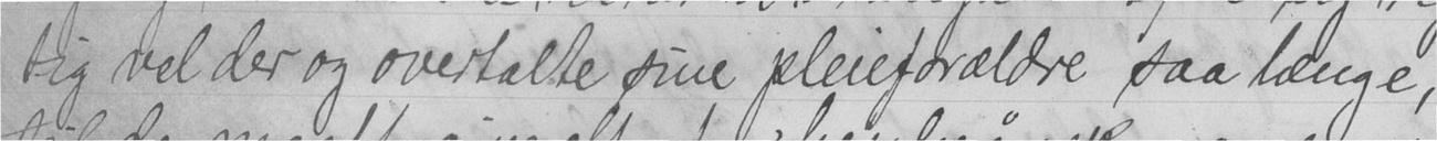tig vel der og overtalte sine pleieforældre saa længe,
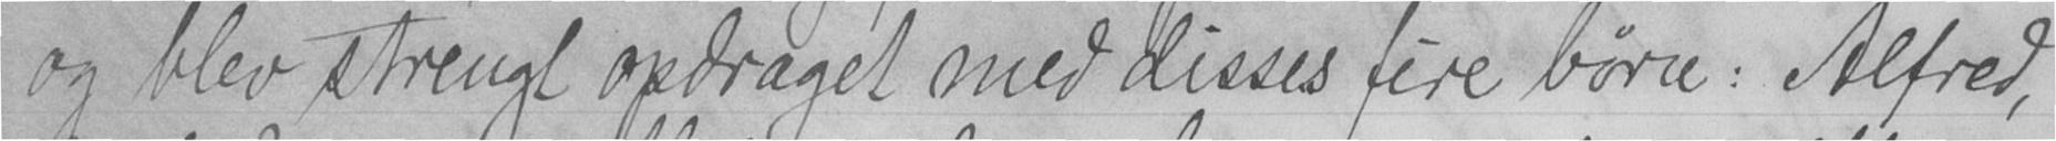og blev strengt opdraget med disses fire børn: Alfred,
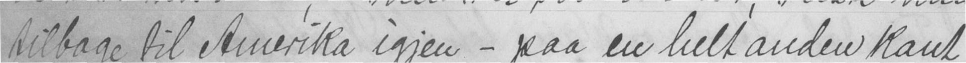tilbage til Amerika igjen - paa en helt anden kant
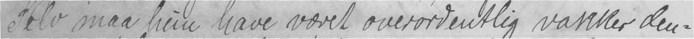Selv maa hun have været overordentlig vakker den-
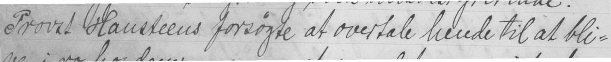Provst Hansteens forsøgte at overtale hende til at bli-
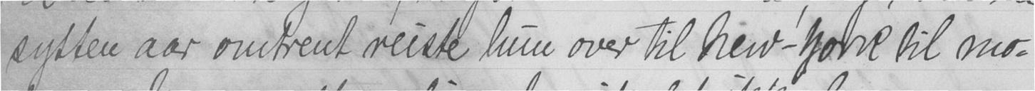sytten aar omtrent reiste hun over til New-York til mo-
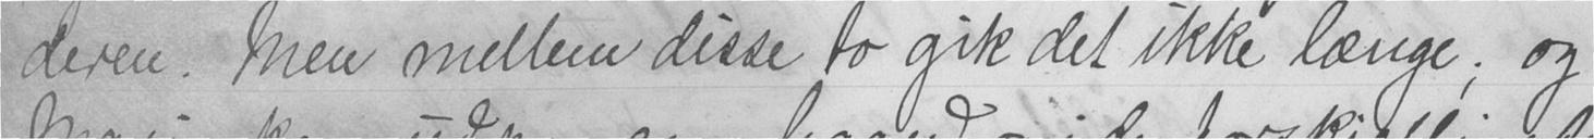deren. Men mellem disse to gik det ikke længe; og
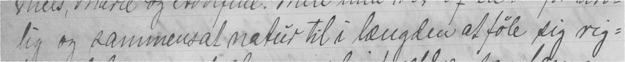lig og sammensat natur til i længden at føle sig rig-
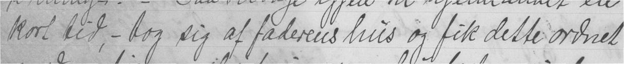kort tid, - tog sig af faderens hus og fik dette ordnet
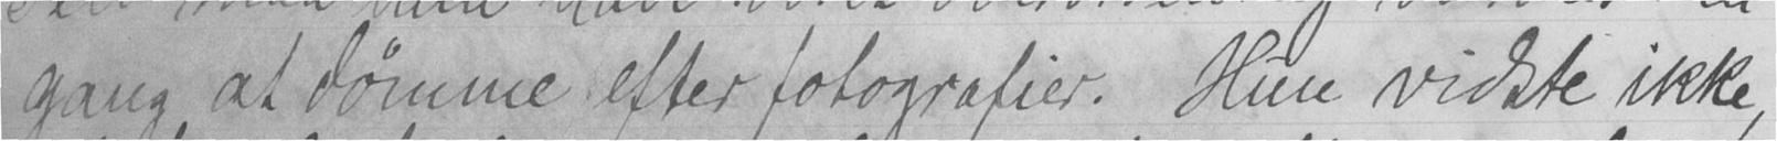gang at dømme efter fotografier. Hun vidste ikke,
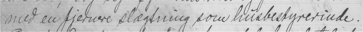med en fjernere slægtning som husbestyrerinde.
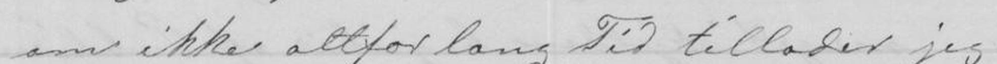om ikke altfor lang Tid tillader jeg
